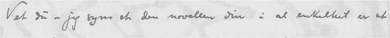Vet du - jeg syns et den novellen din i al enkelket er et
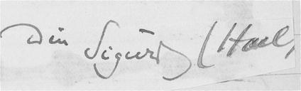Din Sigurd (Hoel
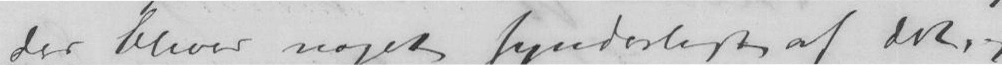der bliver noget synderligt af det, og
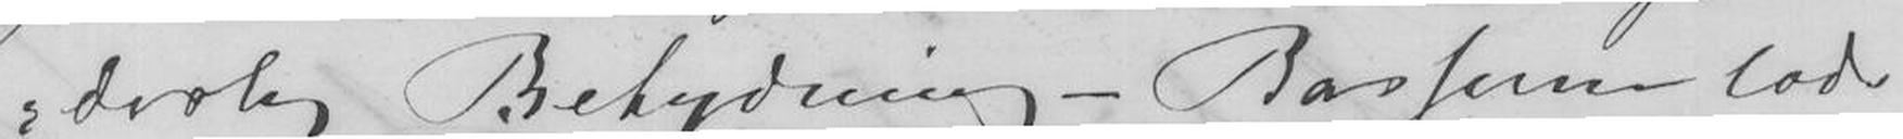derlig Betydning - Basserne lod
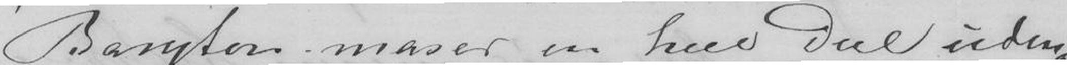Baryton maser en heel Deel uden at
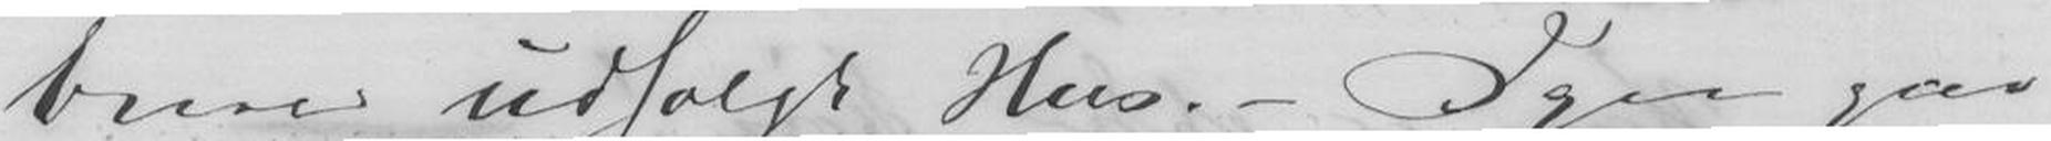bliver udsolgt Hus. - Igen gav
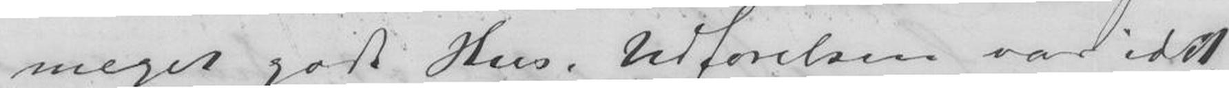meget godt Hus Udførelsen var idet-
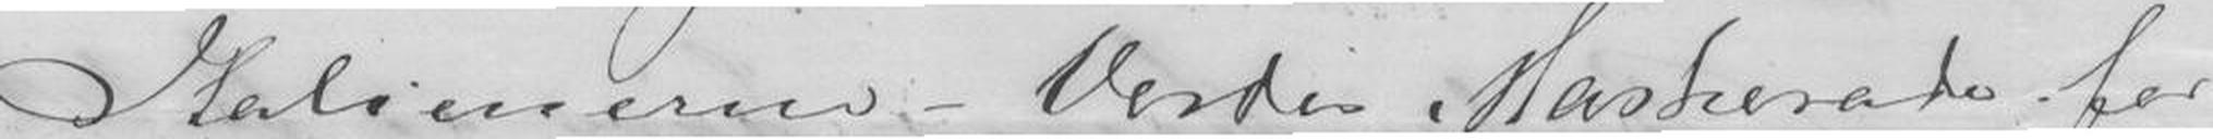Italienerne - Verdis Maskerade for
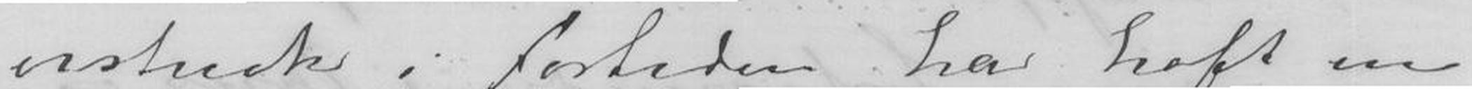vistnok i Fortiden har haft en
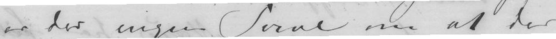er der ingen Tvivl om at der
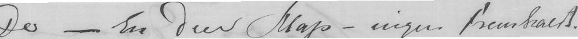De - En Dur Klap - ingen fremkaldt.
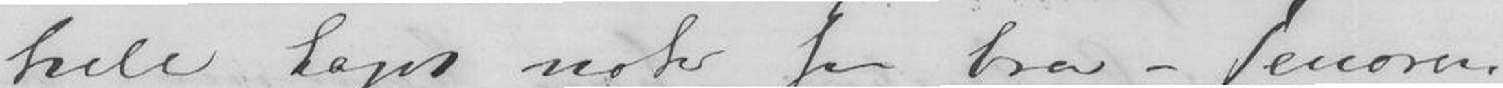hele taget nok saa bra - Tenoren
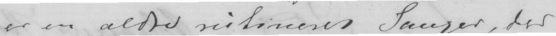er en ældre rutineret Sanger, der
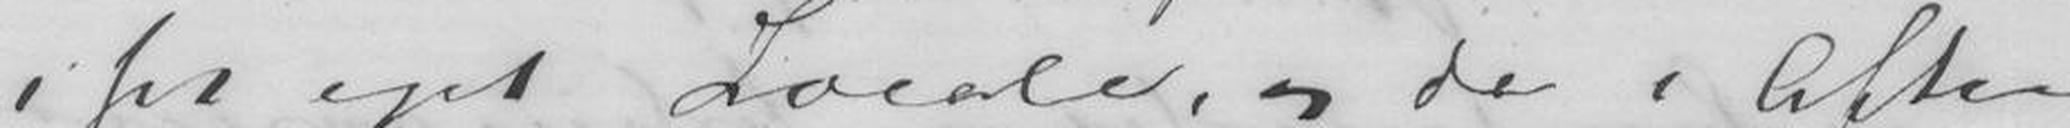i sit eget Locale, og da i Aften
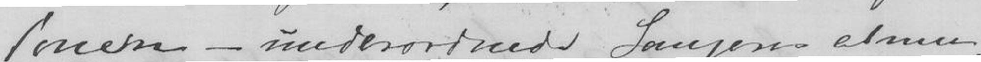Tonerne - underordned Sangens almin-
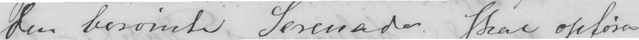den berømte Serenade skal opføres
